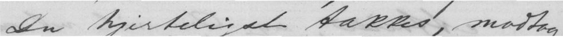Du hjerteligst takkes, modtog
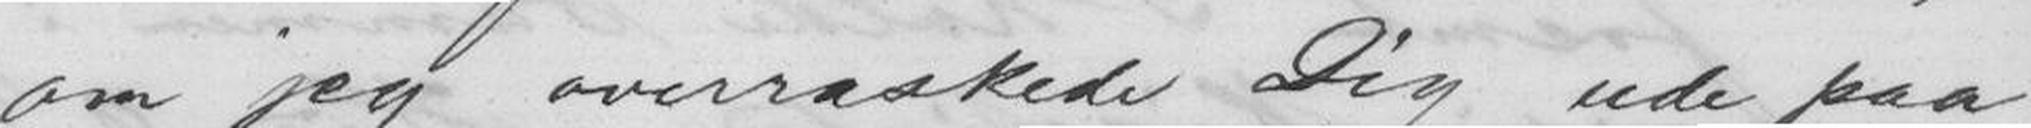om jeg overraskede Dig ude paa
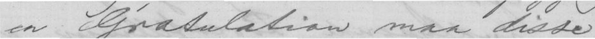en Gratulation maa disse
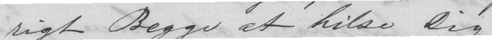rigt Begge at hilse Dig.
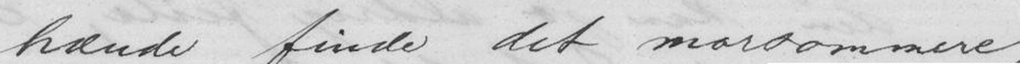hænde finde det morsommere,
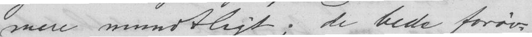mere mundtligt; de bede forøv-
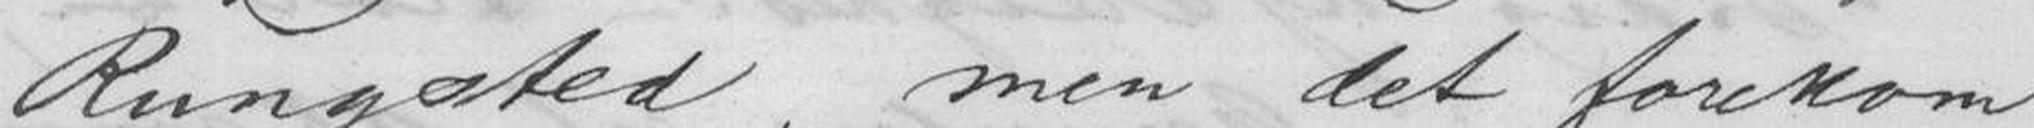Rungsted, men det forekom
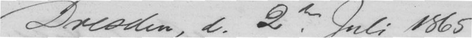Dresden, d. 2den Juli 1865
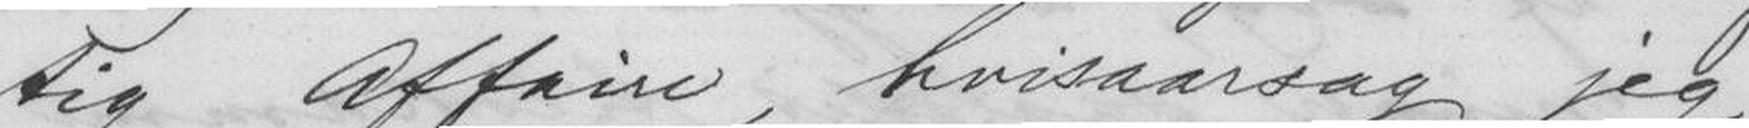tig Affaire, hvisaarsag jeg
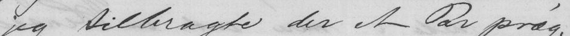jeg tilbragte der et Par præg-
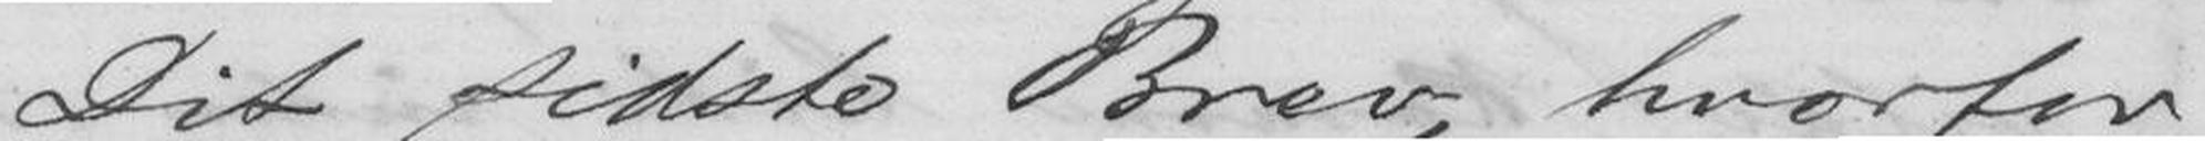Dit sidste Brev, hvorfor
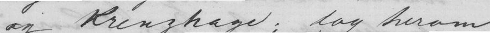og Kreuzhage; dog herom
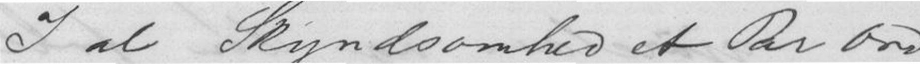I al Skyndsomhed et Par Ord
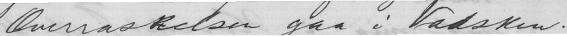Overraskelsen gaa i Vadsken.
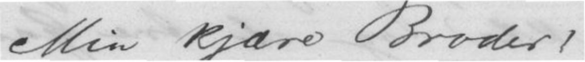Min kjære Broder!
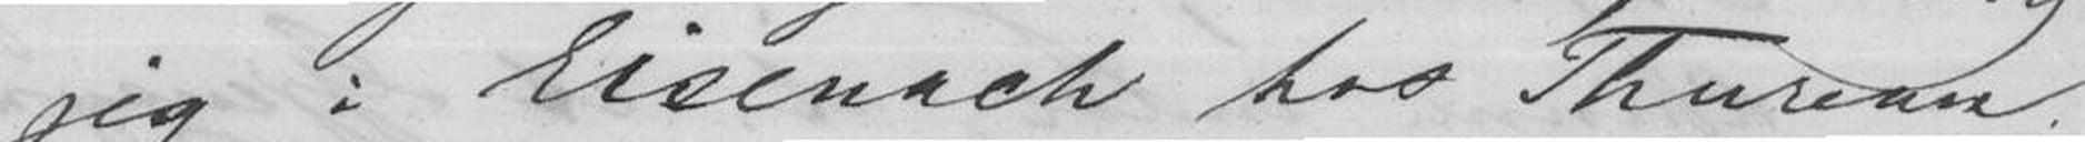jeg i Eisenach hos Thureau;
